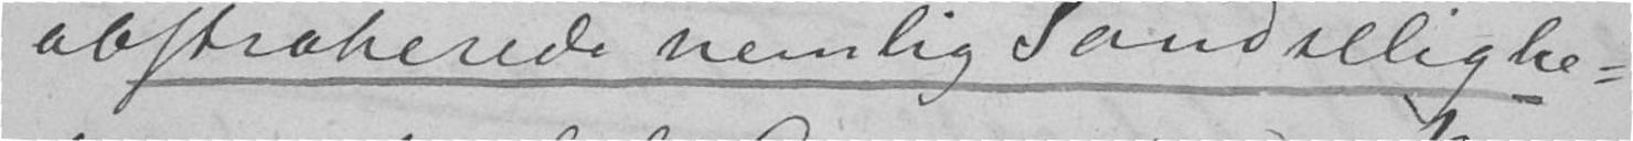abstraherede nemlig Sandselighe-
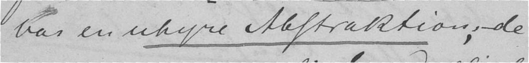har en uhyre Abstraktion; de
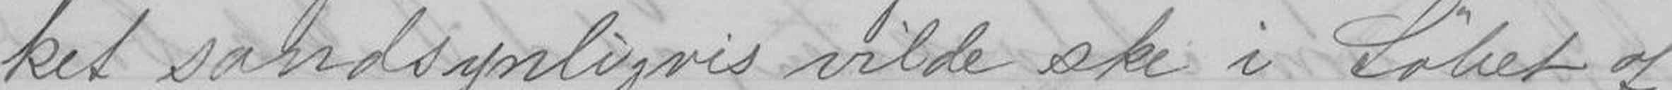ket sandsynligvis vilde ske i Løbet af
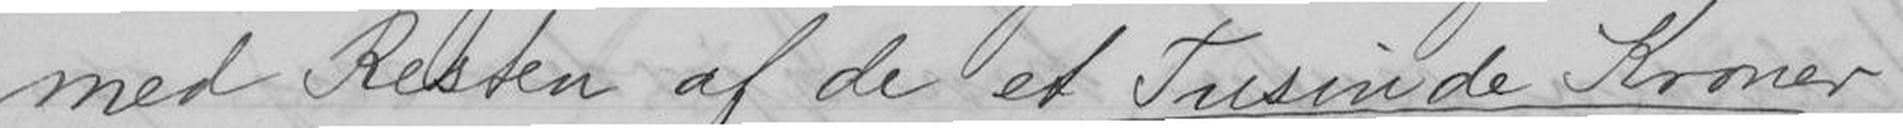med Resten af de et Tusinde Kroner
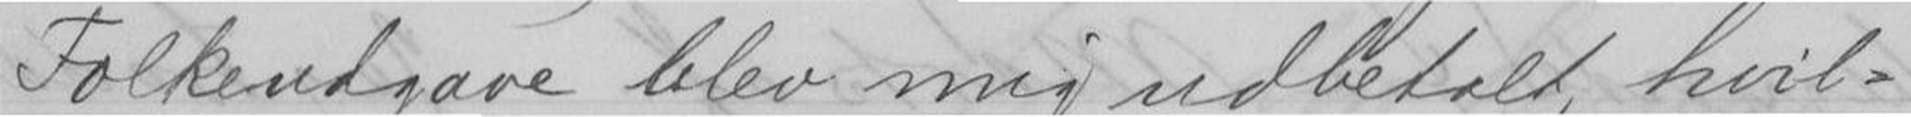Folkeudgave blev mig udbetalt, hvil-
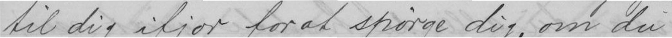til dig ifjor for at spørge dig, om du
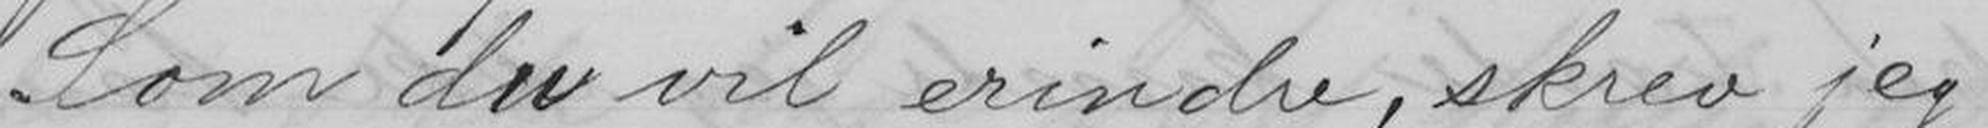Som du vil erindre, skrev jeg
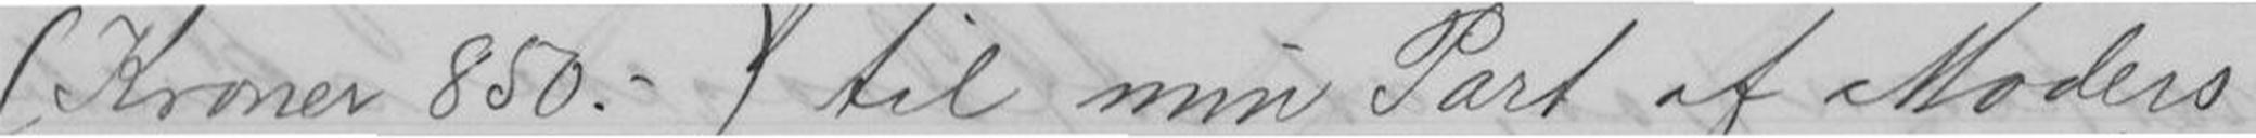(Kroner 850,-) til min Part af Moders
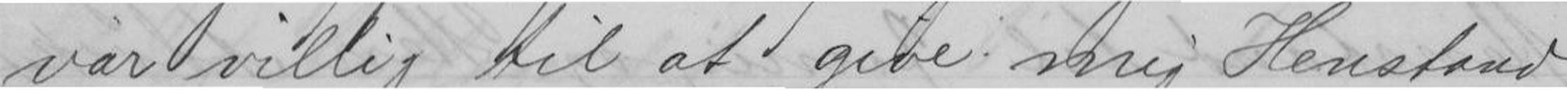var villig til at give mig Henstand
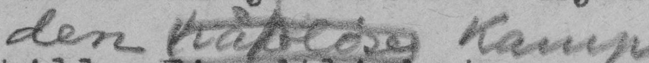den håpløse Kamp
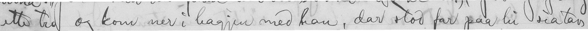ette tro og kom ner i hagjen med han, dar stod far paa hi sia tav
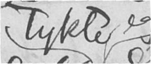tykte eg
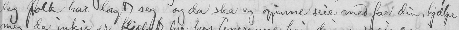leg folk har lagt seg og da ska eg gjenne seie med far din, hjælpe
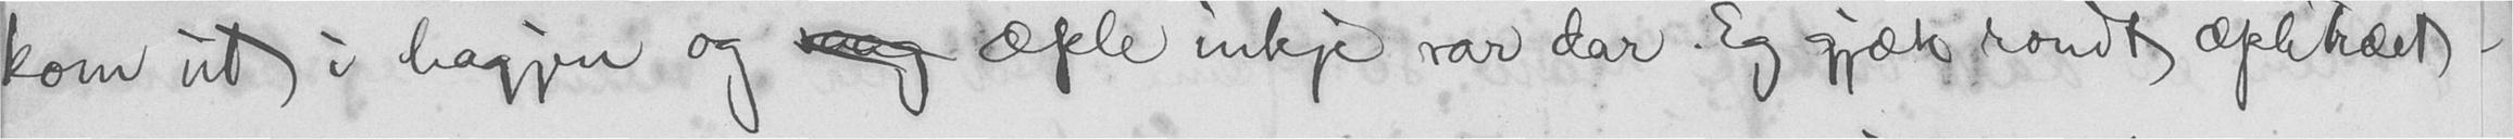kom ut i hagjen, og  æple inkje var dar. Eg gjæk rondt æpletræet
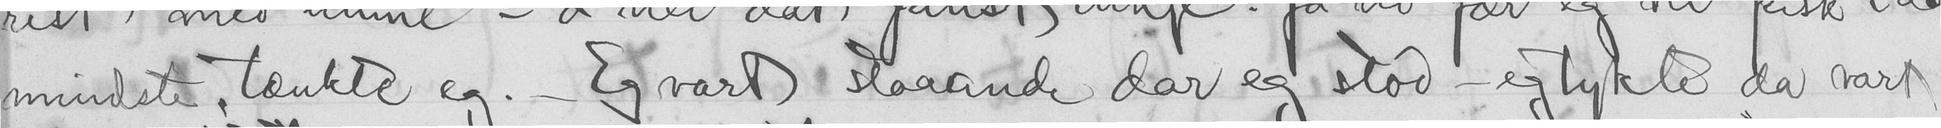mindste, tænkte eg. - Eg vart staaande dar eg stod - eg tykte da vart
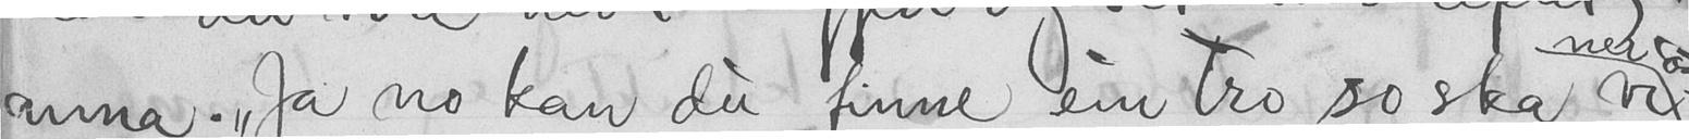anna. "Ja no kan du finne ein tro so ska vi
